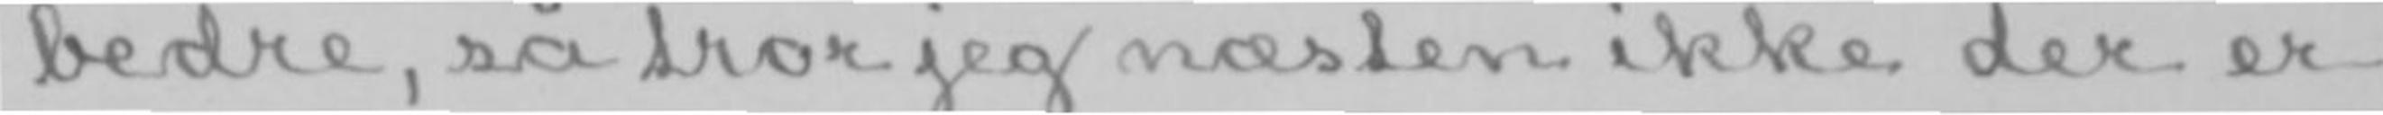bedre, så tror jeg næsten ikke der er
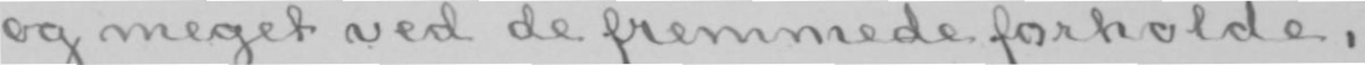og meget ved de fremmede forholde,
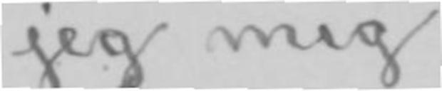jeg mig
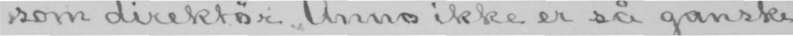som direktør Anno ikke er så ganske
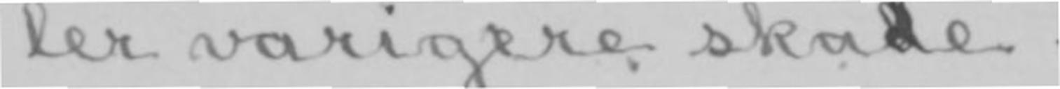ler varigere skade.
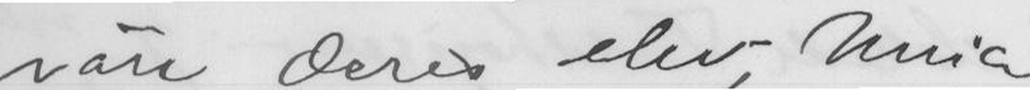väre Deres elev, Nina
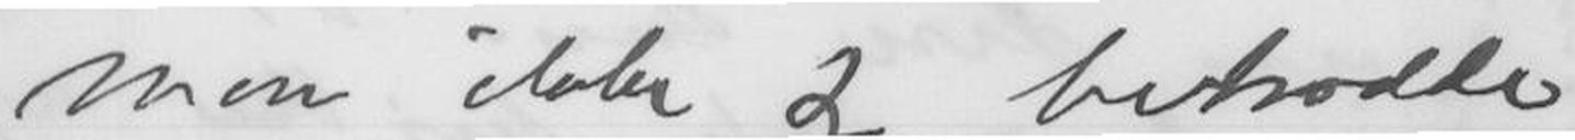Mon ikke jeg betrodde
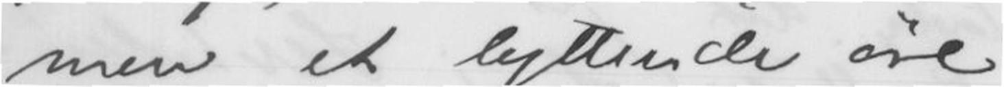men et lyttende öre
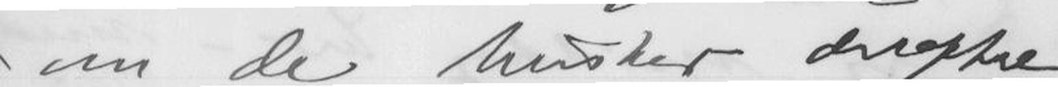om de husker deroppe
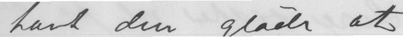havt den gläde at
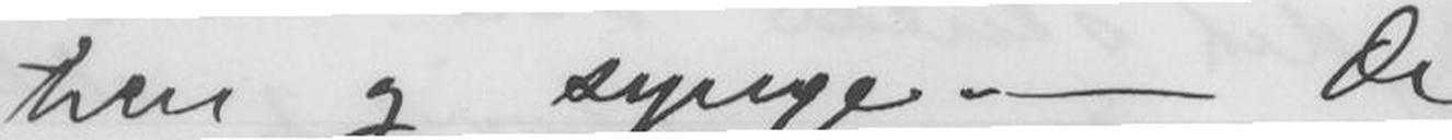hen og synge. - De
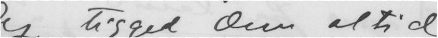Jeg tigget Dem altid
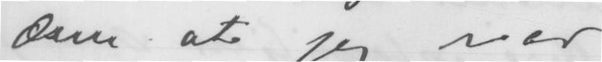Dem at jeg var
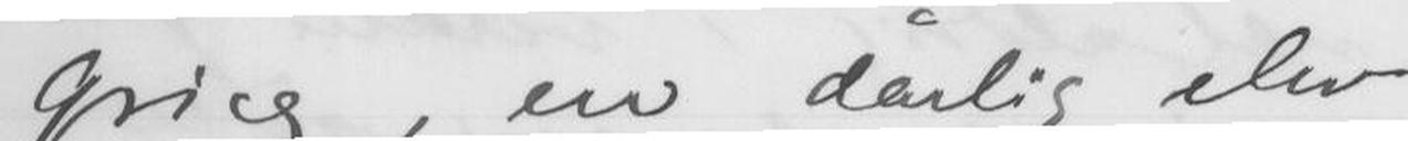Grieg, en dårlig elev,
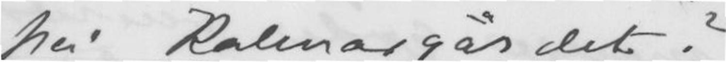på Kalmergärdet?
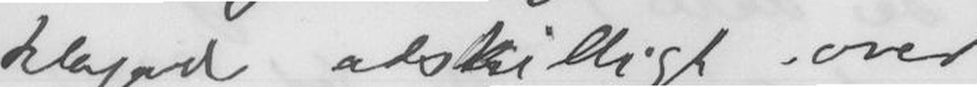klaged adskilligt over
